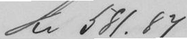kr 581.87
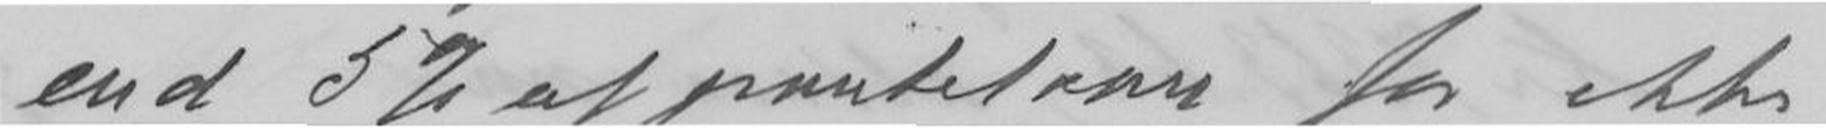end 5% af pantelaan for ikke
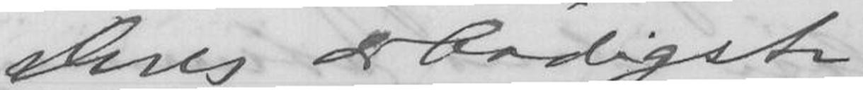Deres ærbødigste
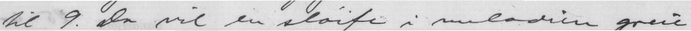til 9. Da vil en sløife i melodien greie
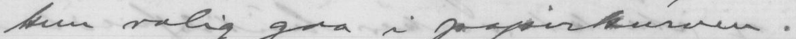kun rolig gaa i papirkurven.
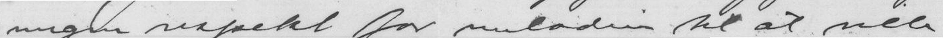megen respekt for melodien til at selv
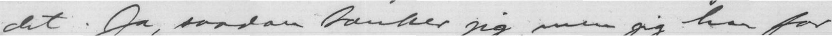det. Ja, saadan tænker jeg, men jeg har for
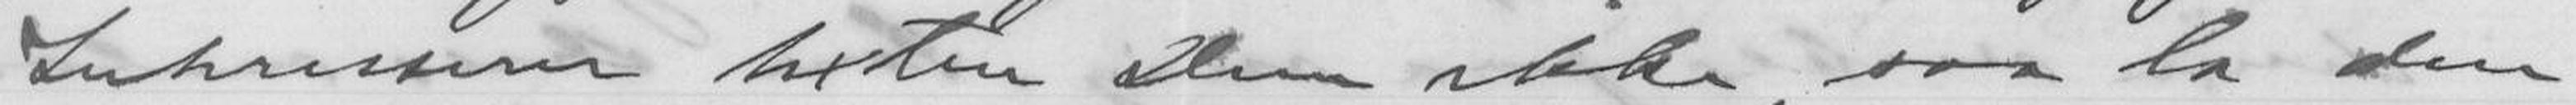Interesser texten Dem ikke, saa la den
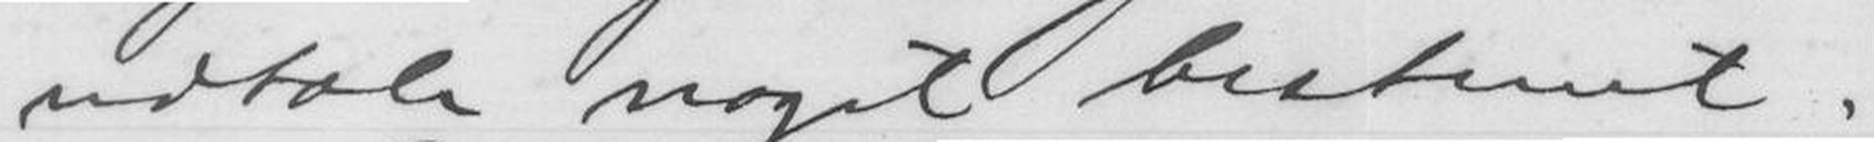udtale noget bestemt.
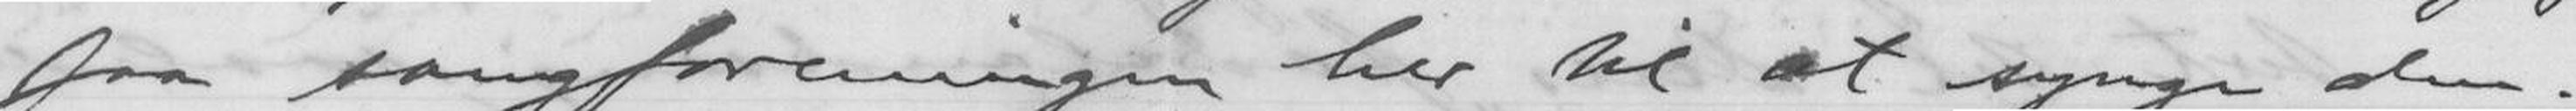faa sangforeningen her til at synge den.
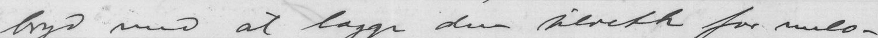bryd med at lægge den tilrette for melo-
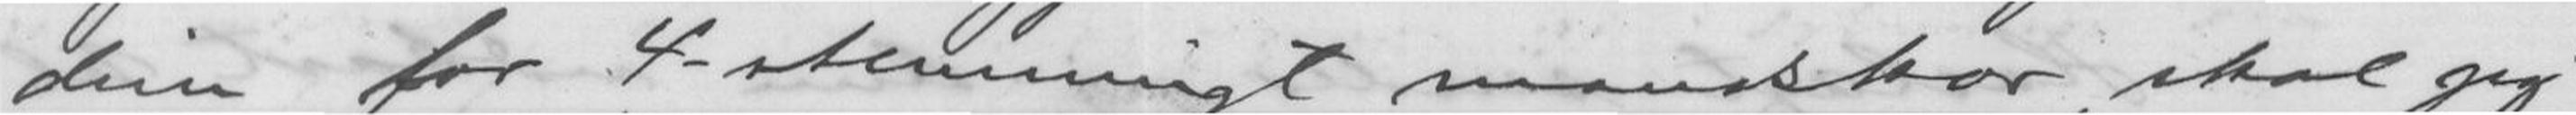dien for 4-stemmigt mandskor, skal jeg
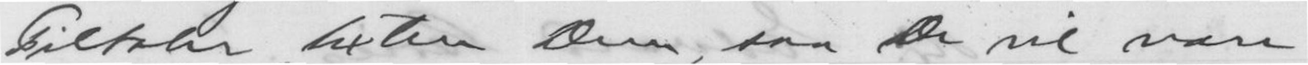Tiltaler texten Dem, saa De vil være
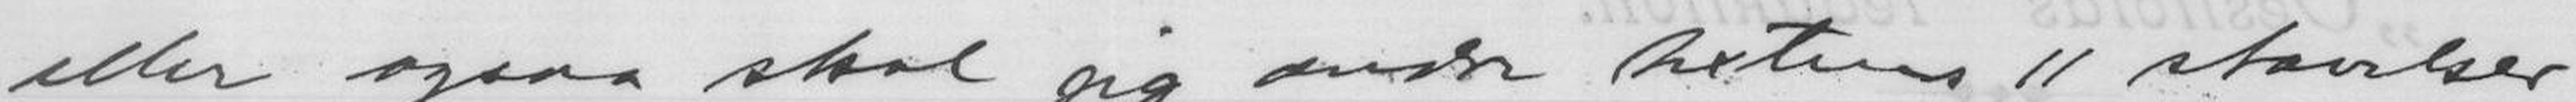eller ogsaa skal jeg ændre textens 11 stavelser
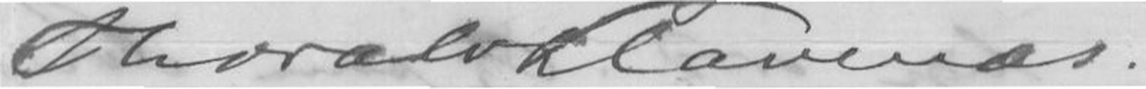Thorvald Klavenæs.
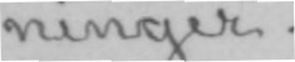ninger.
